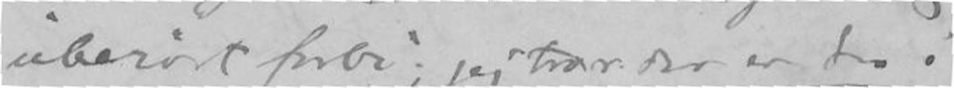uberørt forbi; jeg tror der er tro i
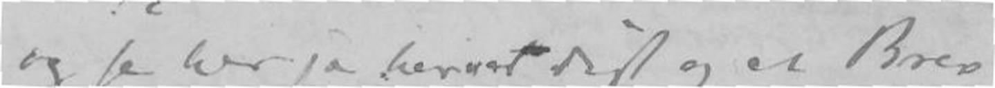og se her ja her er et digt og et Brev
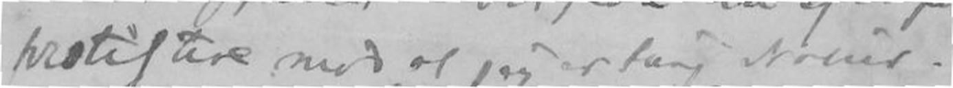tilstistere med at jeg er tung Natur.
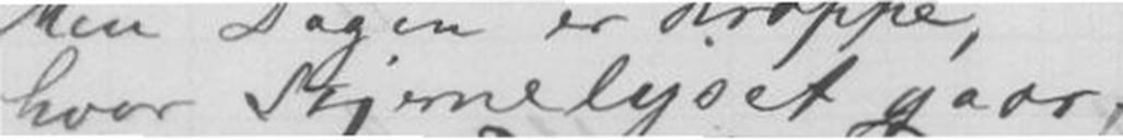hvor Stjernelyset gaar,
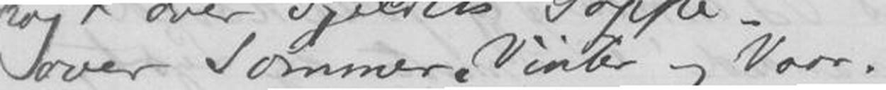over Sommer, Vinter og Vaar.
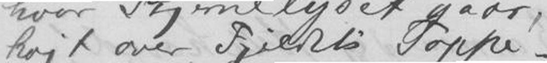højt over Fjeldets Toppe,
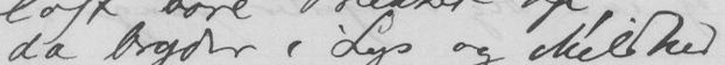da bryder i Lys og Mildhed
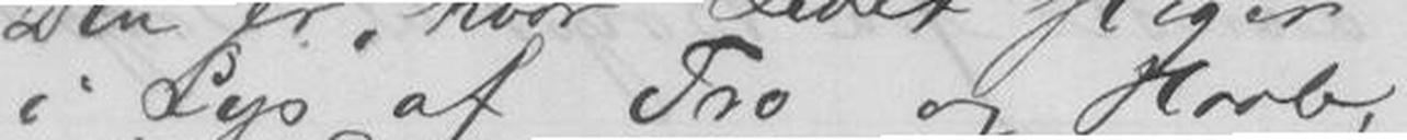i Lys af Tro og Haab,
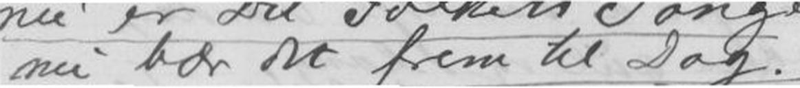nu leder det frem til Dag.
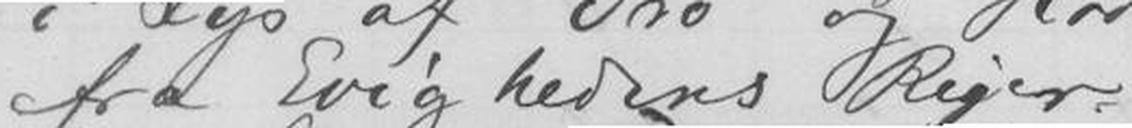fra Evighedens Riger.
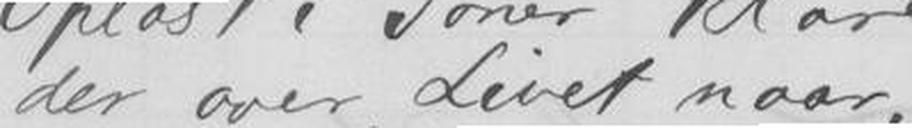der over Livet naar,
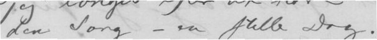den Song - en stille Dag.
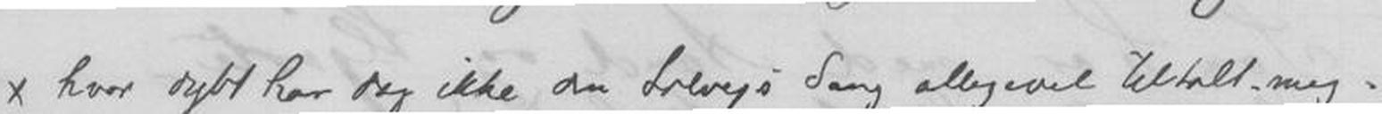x hvor dybt dog ikke den Solveigs Sang Alligevel tiltalt mig.
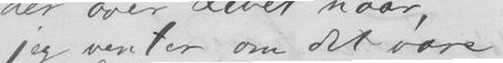jeg venter om det vare
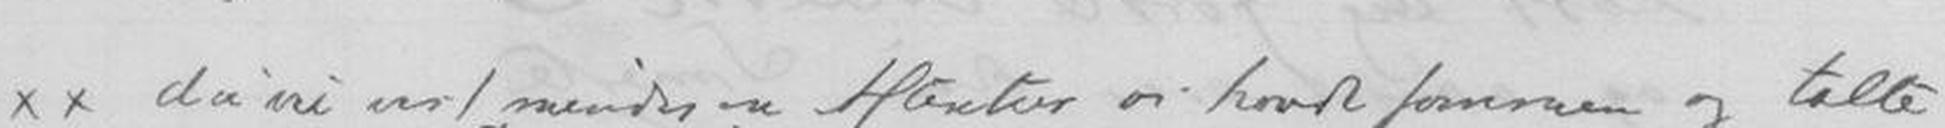xx du vil vist mindes en Aftentur vi havde sammen og Talte
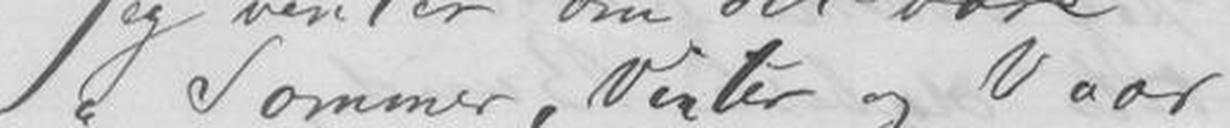o Sommer, Vinter og Vaar
